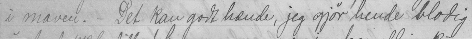i maven. - Det kan godt hænde, jeg gjør hende blodig
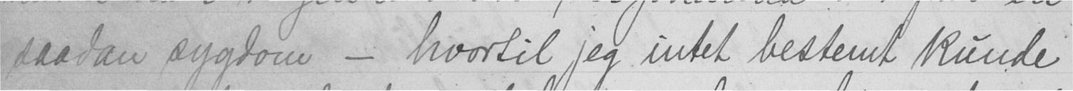saadan sygdom - hvortil jeg intet bestemt kunde
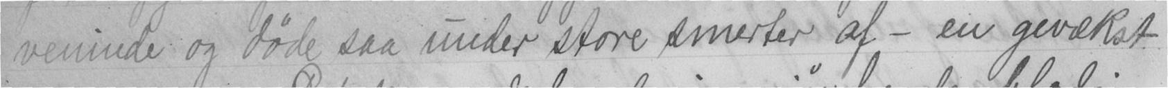veninde og døde saa under store smerter af - en gevækst
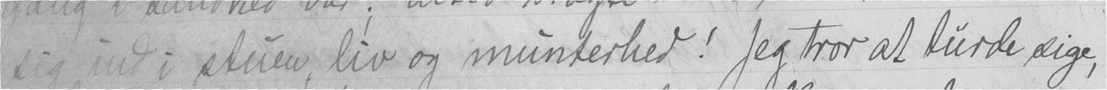sig ind i stuen, liv og munterhed! Jeg tror at turde sige,
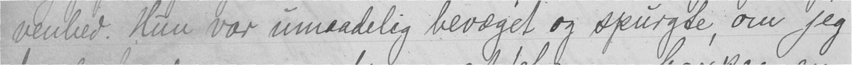venhed. Hun var umaadelig bevæget og spurgte, om jeg
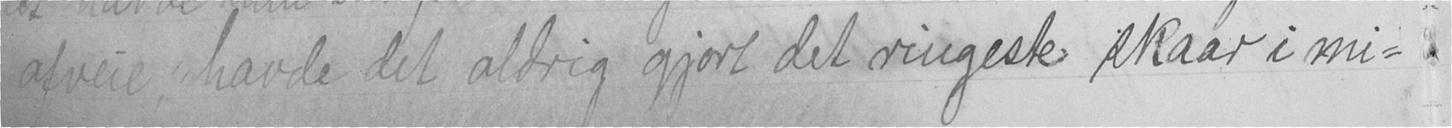afveie, havde det aldrig gjort det ringeste skaar i mi-
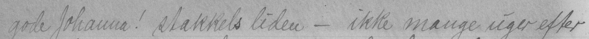gode Johanna! stakkels liden - ikke mange uger efter
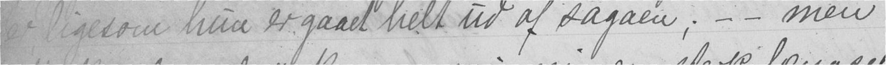er ligesom hun er gaaet helt ud af sagaen; - - men
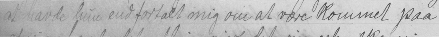at havde hun end fortalt mig om at være kommet paa
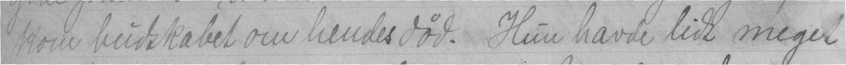kom budskabet om hendes død. Hun havde lidt meget
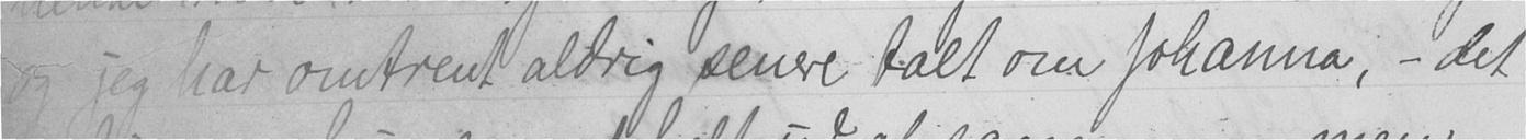og jeg har omtrent aldrig senere talt om Johanna, - det
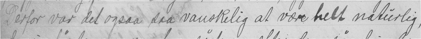Derfor var det ogsaa saa vanskelig at være helt naturlig,
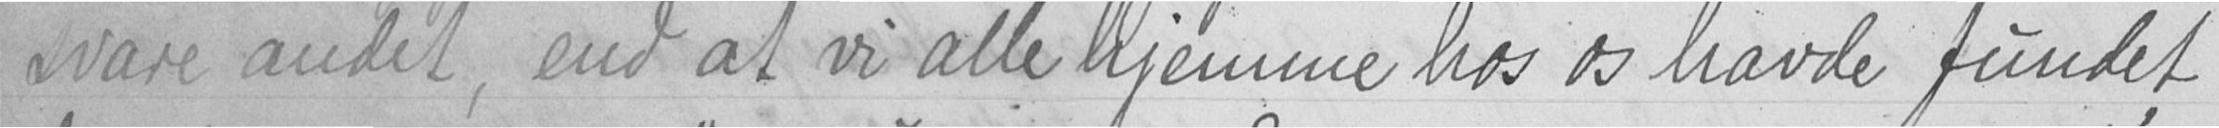svare andet, end at vi alle hjemme hos os havde fundet
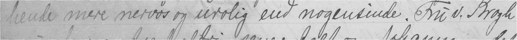hende mere nervøs og urolig end nogensinde. Fru v. Krrogh
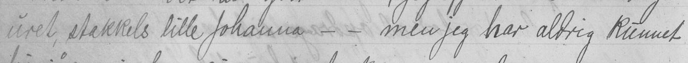uret, stakkels lille Johanna - - men jeg har aldrig kunnet
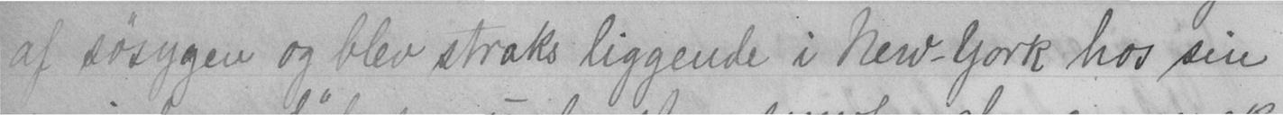af søsygen og blev straks liggende i New-York hos sin
